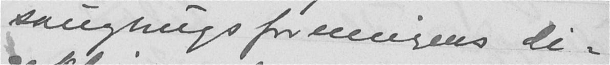saugbrugsforeningens di-
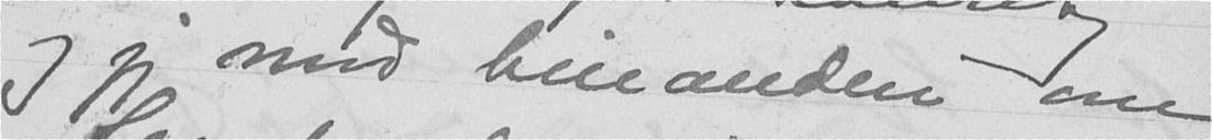og jeg mod hinanden om
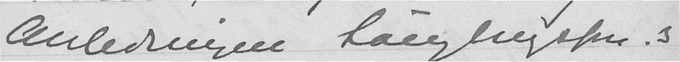Anledningen Saugbrugsforn.s
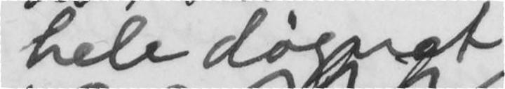hele døgnet
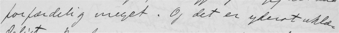forfærdelig meget. Og det er yderst uklæ-
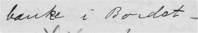banke i Bordet
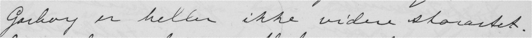Garborg er heller ikke videre storartet.
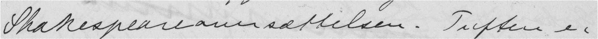Shakespeareoversættelsen. Tuften er
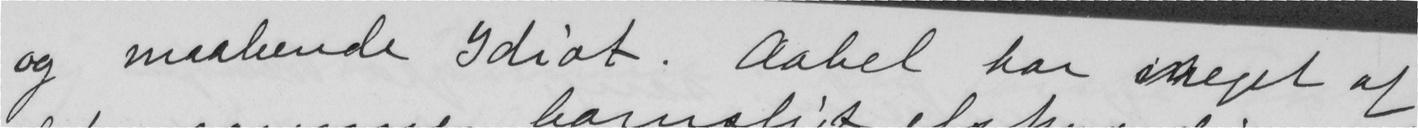og maabende Idiot. Aabel har seget af
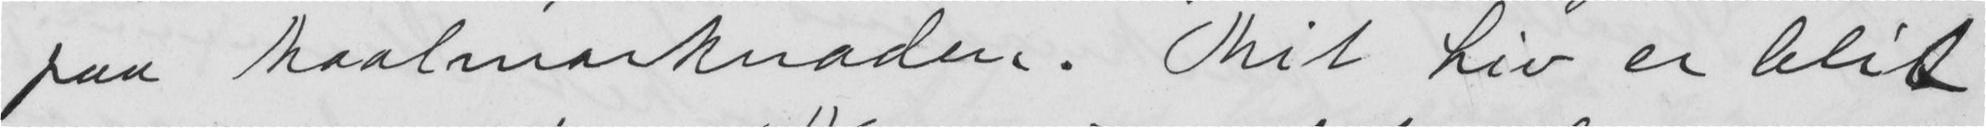paa Maalmarknaden. Mit Liv er blit
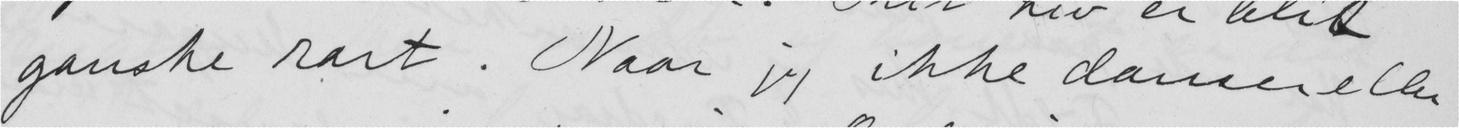ganske rart. Naar jeg ikke danser eller
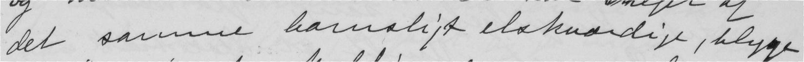det samme barnsligt elskværdige, blyge
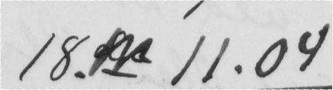18. 11.04
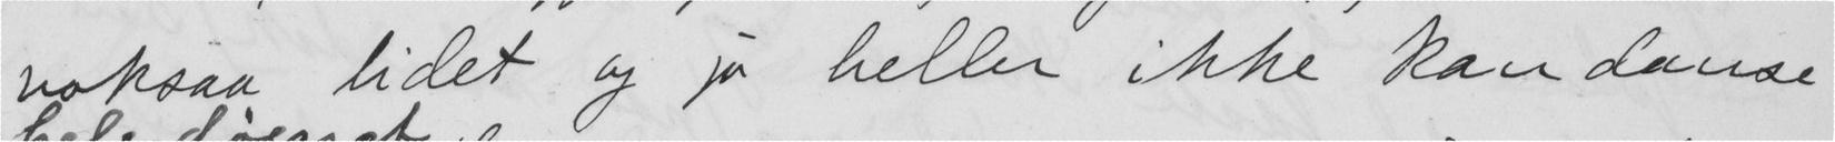noksaa lidet og jo heller ikke kan danse
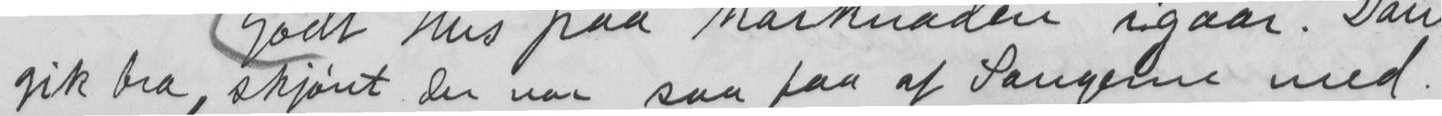gik bra, skjønt der var saa faa af Sangerne med.
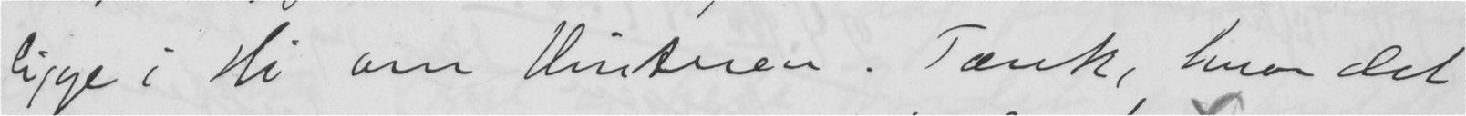ligge i Hi om Vinteren. Tænk, hvor det
